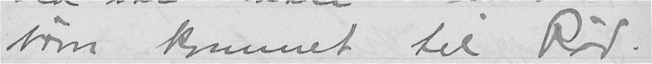børn kommet til Rød.
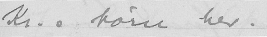Kr.s børn her.
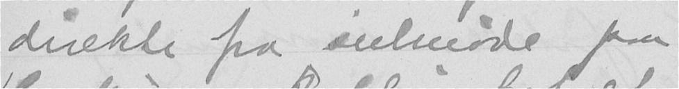direkte fra seilmøde paa
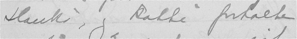Hankø, og Katti fortalte
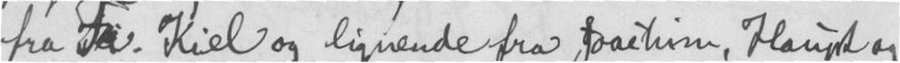fra Fr. Kiel og Lignende fra Joachim, Haugst og
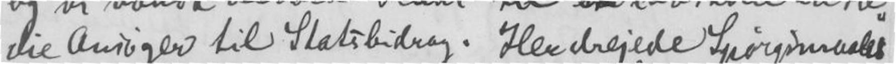die Ansøger til Statsbidrag. Her drejede Spørgsmaalet
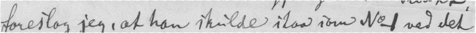foreslog jeg, at han skulde staa som No 1 ved det
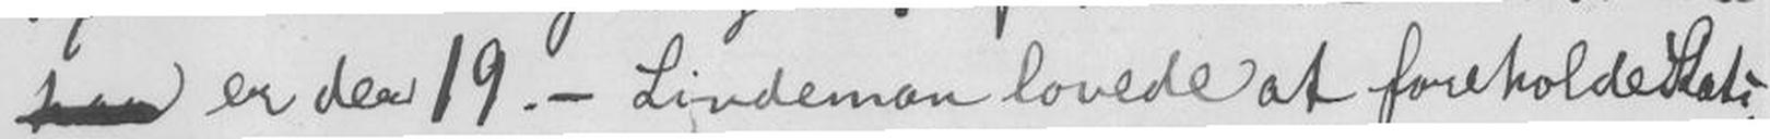er der 19. - Lindeman lovede at foreholde Stats-
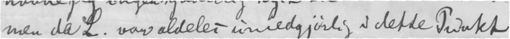men da L. var aldeles umedgjørlig i dette Punkt,
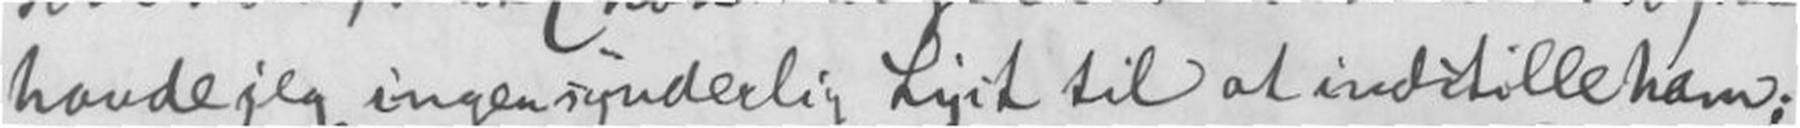havde jeg ingen synderlig Lyst til at indstille ham;
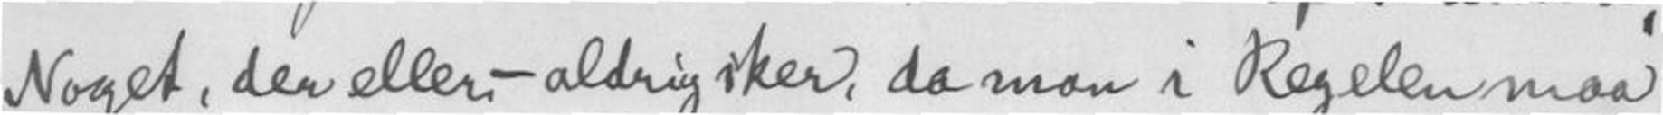Noget, der eller - aldrig sker, da man i Regelen maa
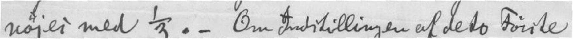nøjes med 1/3. - Om Indstillingen af de to Første
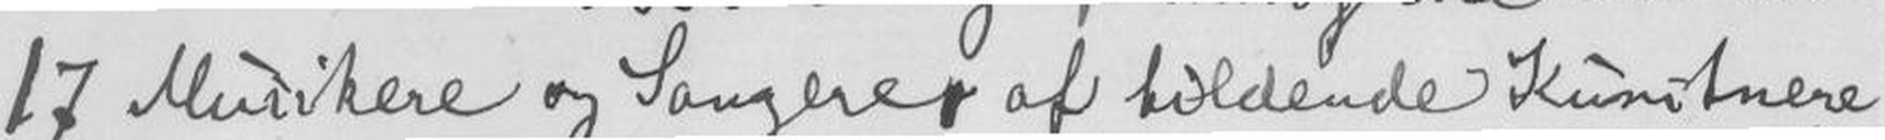17 Musikere og Sangere, af bildende Kunstnere
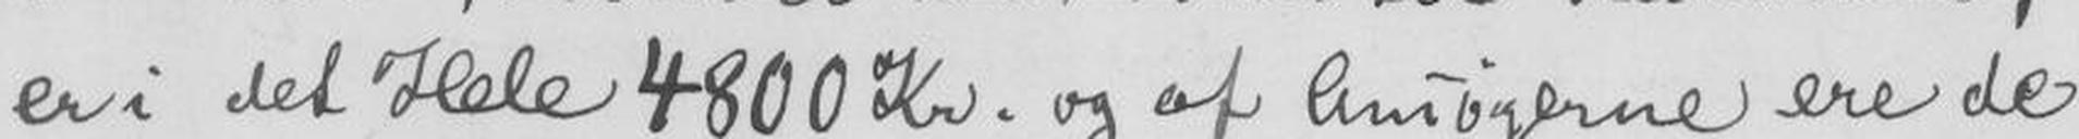er i det Hele 4800 Kr. og af Ansøgerne ere de
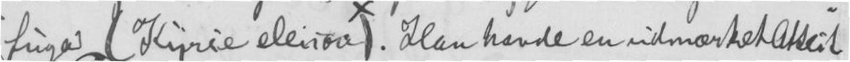fuge (Kyrie eleison). Han havde en udmærket Attest
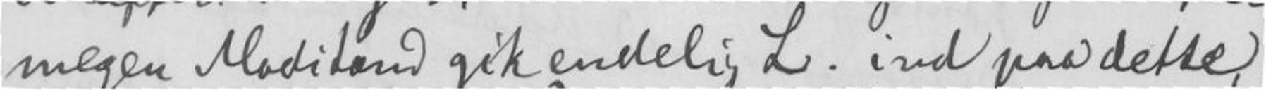megen Modstand gik endelig L. ind paa dette,
